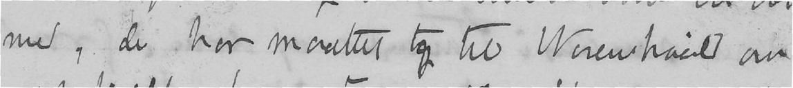med, de har maattet ty til Werenskiold om
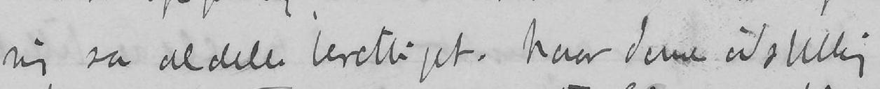mig da aldeles berettiget. Naar denne udstilling
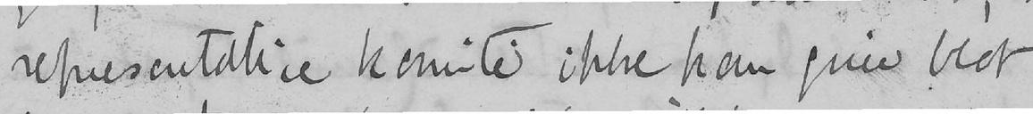representative komité ikke kan greie blot
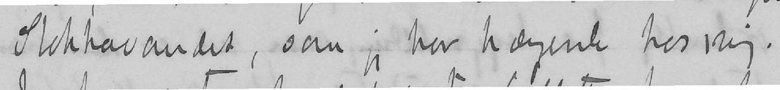Stokkavandet, som jeg har hængende hos mig.
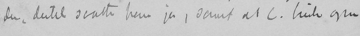der, dertil svarte han ja, samt at C. burde ogsaa
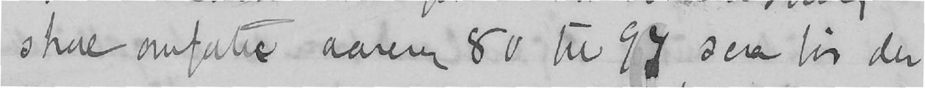skal omfatte aarene 80 til 97 saa bør der
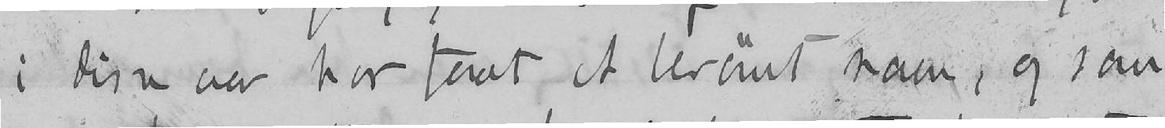i disse aar har faat et berømt navn, og som
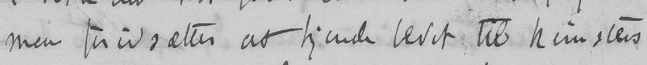man forudsætter at kjende bedst til kunstens
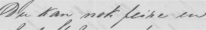Du kan nok feire en
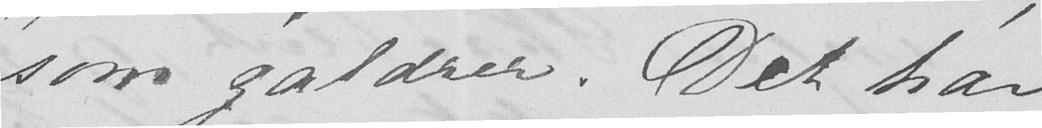som galdrer. Det har
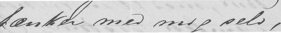tænker med mig selv,
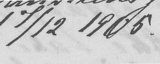17/12 1905.
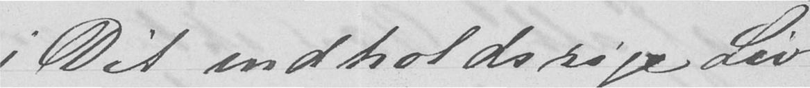i Dit indholdsrige Liv
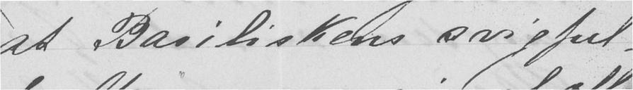at Basiliskens svigful-
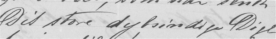Dit store dybsindige Digt
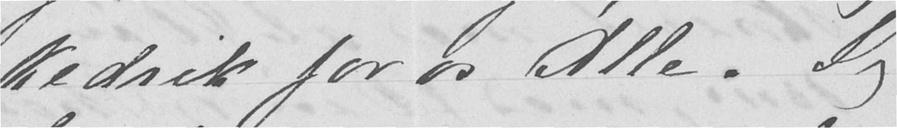kedrik for os Alle. Jeg
